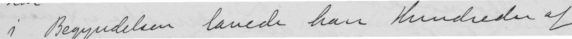i Begyndelsen lavede han Hundreder af
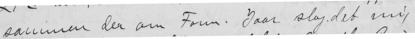sammen der om Form. Iaar slog det mig
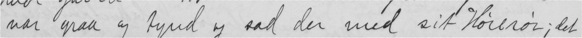var graa og tynd og sad der med sit Hørerør; det
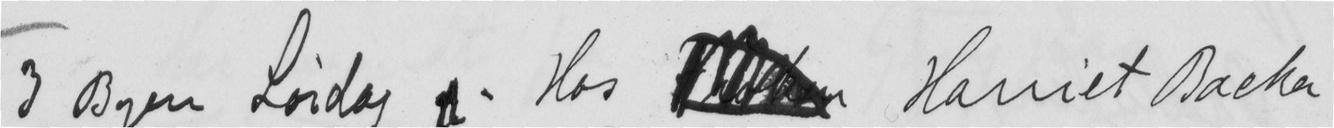I Byen Lørdag  Hos  Harriet Backer
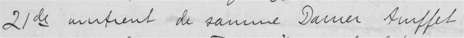21de omtrent de samme Damer truffet
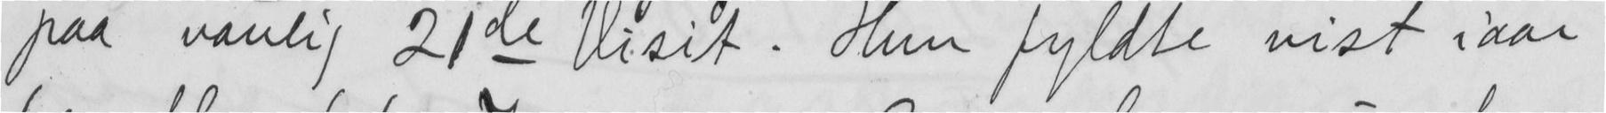paa vanlig 21de Visit. Hun fyldte vist iaar
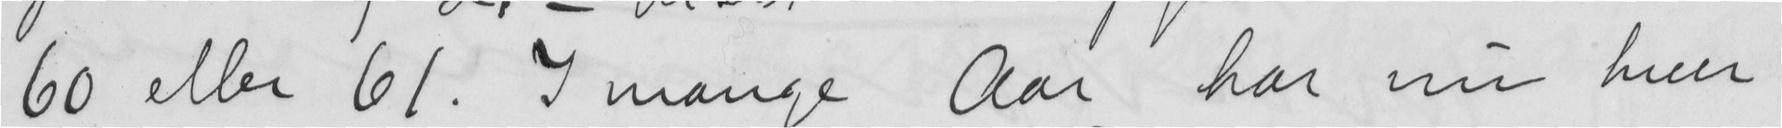60 eller 6l. I mange Aar har nu hun
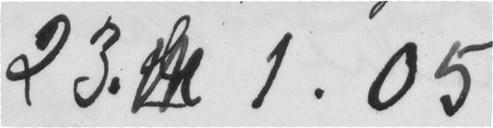23. 1.05
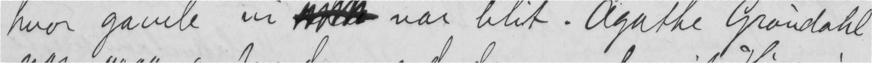hvor gamle vi  var blit. Agathe Grøndahl
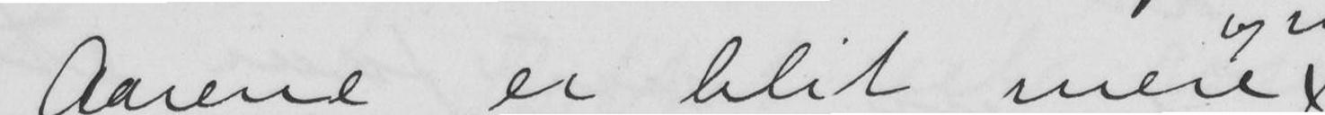Aarene er blit mere
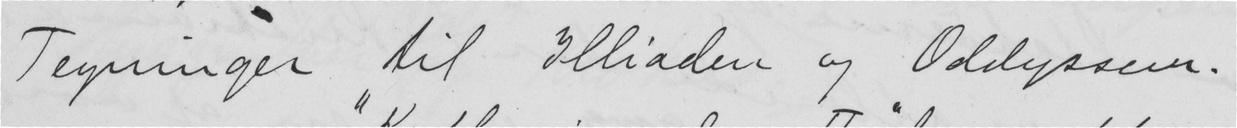Tegninger til Illiaden og Oddysseen.
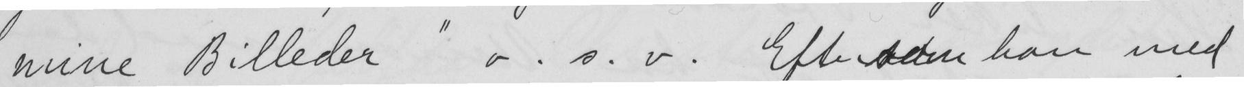mine Billeder" o. s. v. Efter han med
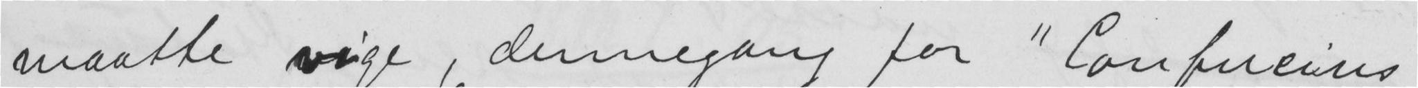maatte vige, dennegang for "Confueins
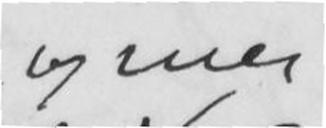og mer
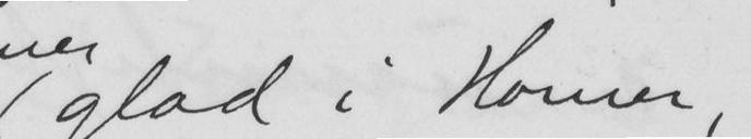glad i Homer,
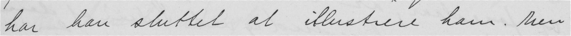har han sluttet at illustrere ham. Men
